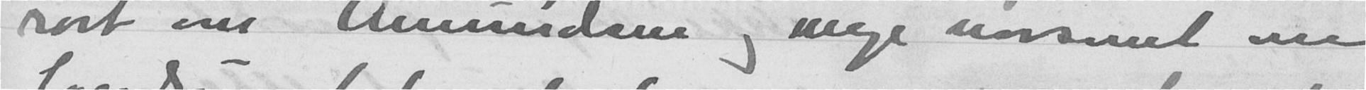rart om Amundsen og mye morsomt om
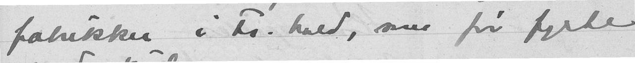fabrikker i Fr. hald, som før fyrte
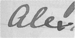Alex
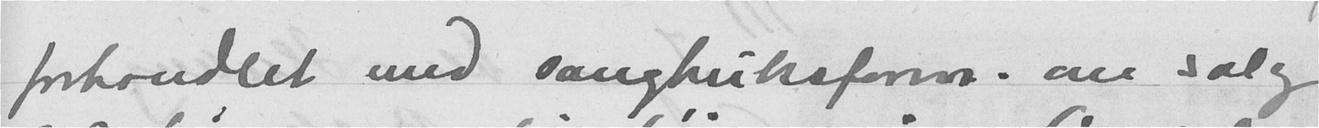forhandlet med saugbruksforn. om salg
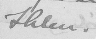Helen.
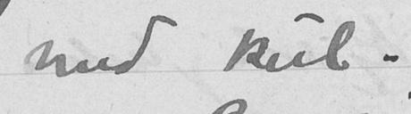med kul.
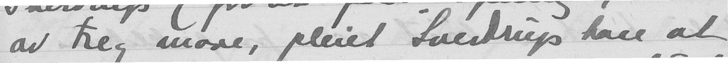av treg mave, pleiet Sverdrup han at
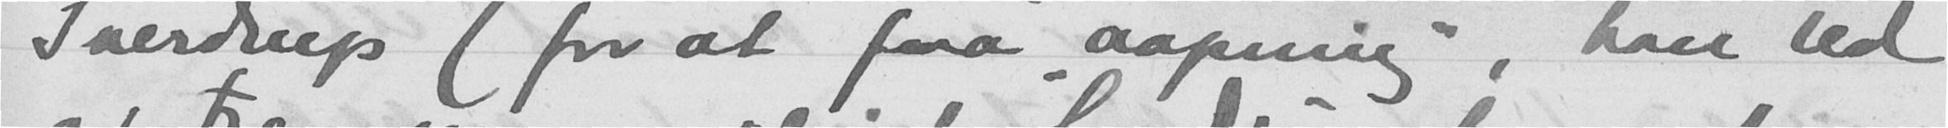Sverdrup (for at faa aapning, han led
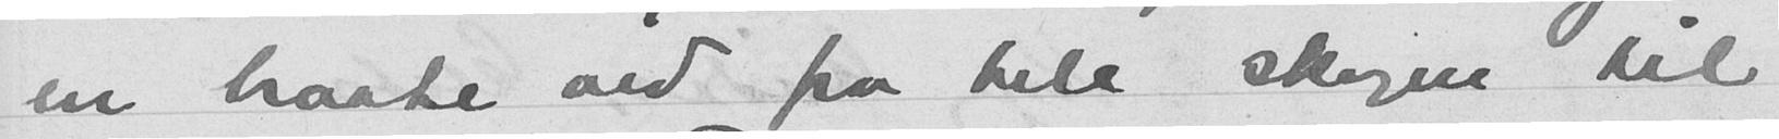en braate ved fra hele skogen til
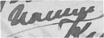Nansen
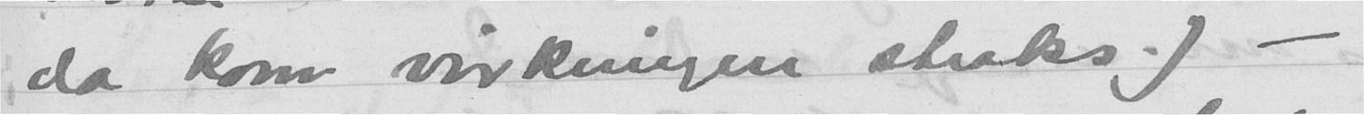da kom virkningen straks.) -
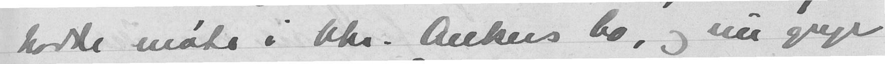hadde møte i Chr. Ankers bo, og nu gryer
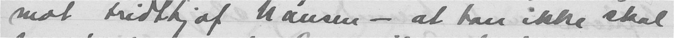mot Fridtjof Nansen - at han ikke skal
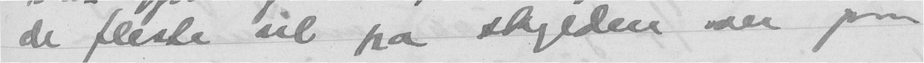de fleste vil ha skylden mer paa
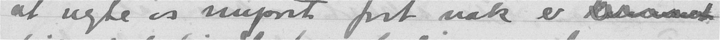at negte os import fort nok er konant
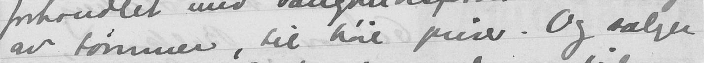av tømmer, til høie priser. Og sælger
